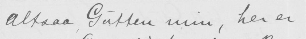Altsaa Gutten min, her er
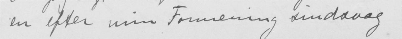en efter min Formening sindsvag
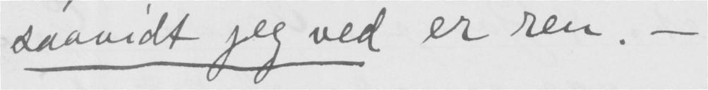saavidt jeg ved er ren. —
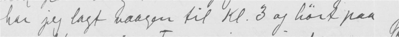har jeg lagt vaagen til Kl. 3 og hørt paa
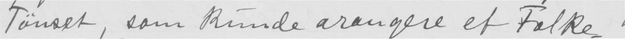Tønset, som Kunde arangere et Folke-
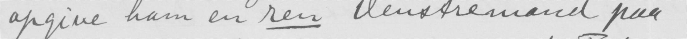opgive ham en ren Venstremand paa
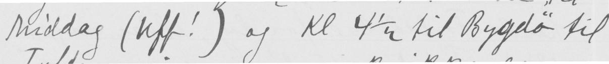Middag (uff!) og Kl 4½ til Bygdø til
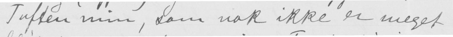Tuften min, som nok ikke er meget
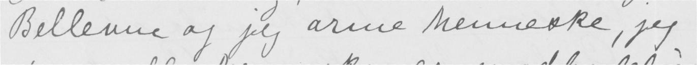Bellevue og jeg arme Menneske, jeg
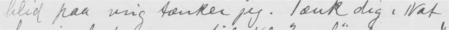blid paa mig tænker jeg. Tænk dig i Nat
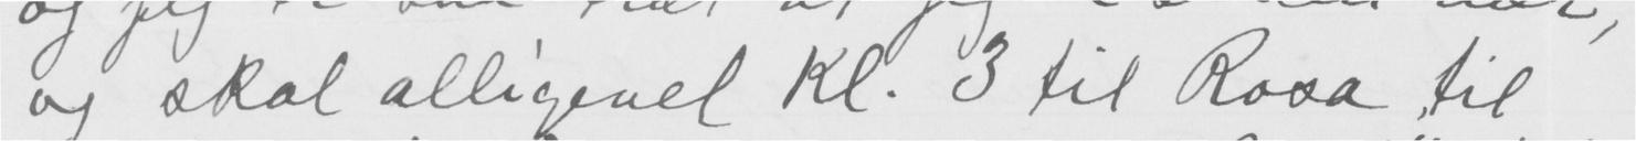og skal alligevel Kl. 3 til Rosa til
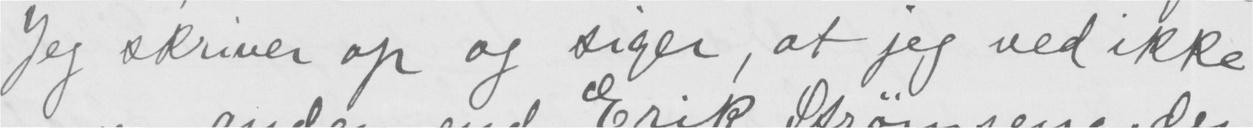Jeg skriver op og siger, at jeg ved ikke
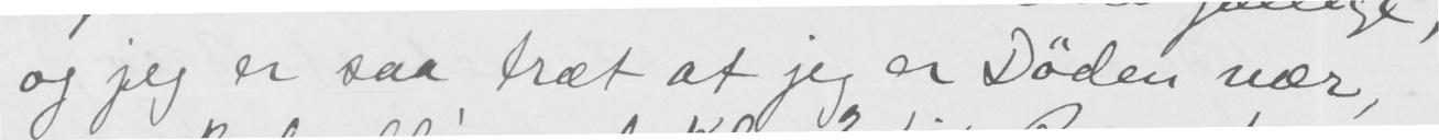og jeg er saa træt at jeg er Døden nær,
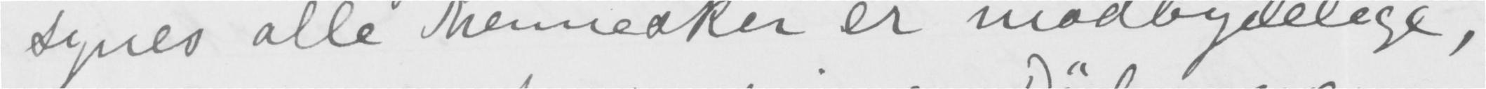synes alle Menesker er modbydelige,
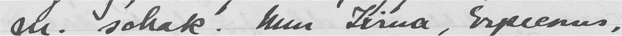m. schak. Men Dina, Erpcenus,
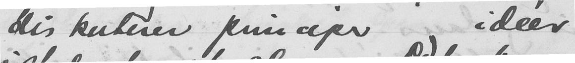diskuturer prisecipe idéer
